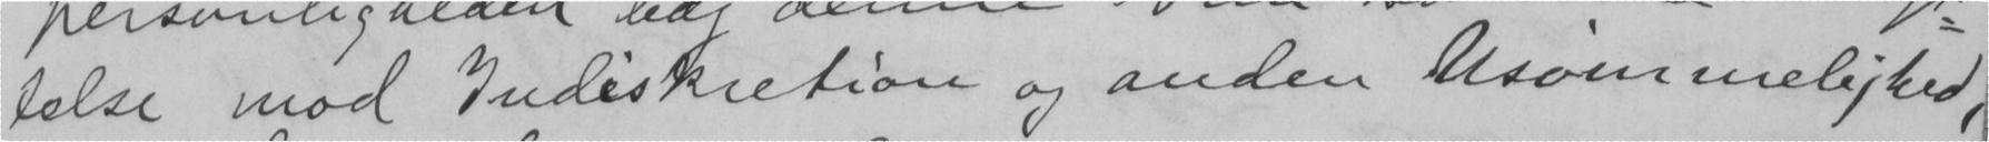telse mod Indiskretion og anden Usømmelighed,
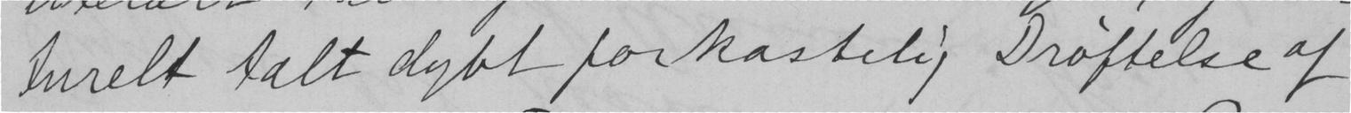turelt talt dybt forkastelig Drøftelse af
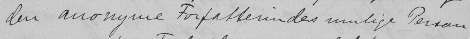den anonyme Forfatterindes mulige Person
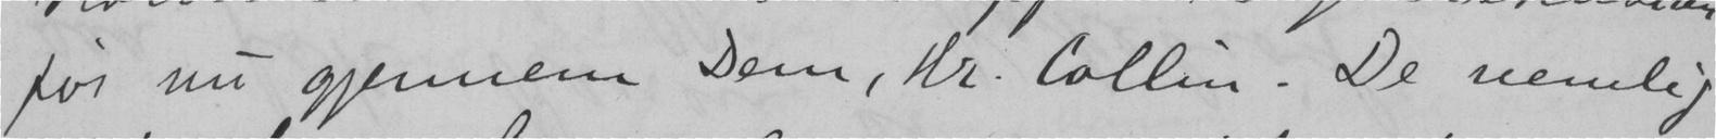før nu gjennem Dem, Hr. Collin. De nemlig
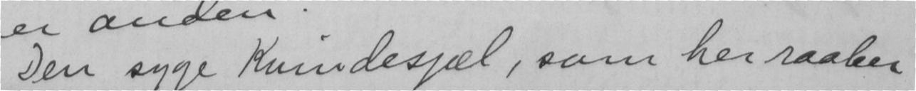Den syge Kvindesjæl, som her raaber
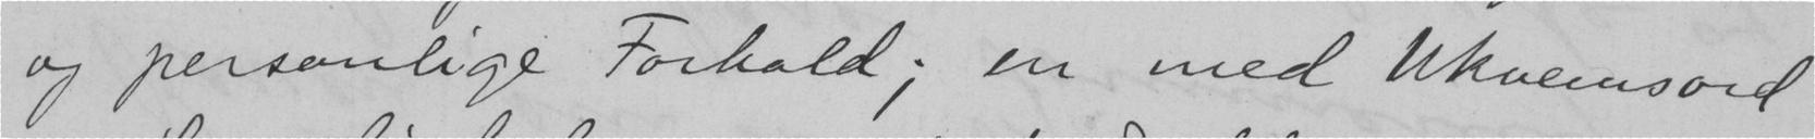og personlige Forhold; en med Ukvemsord
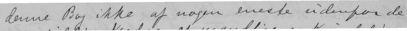denne Bog ikke af nogen eneste udenfor de
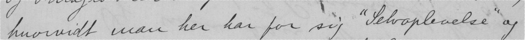hvorvidt man her har for sig "Selvoplevelse" og
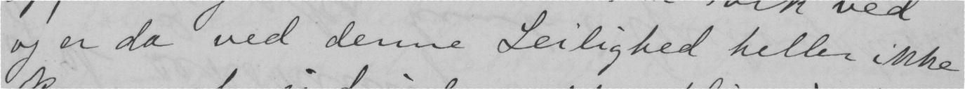og er da ved denne Leilighed heller ikke
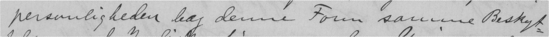personligheden bag denne Form samme Beskyt-
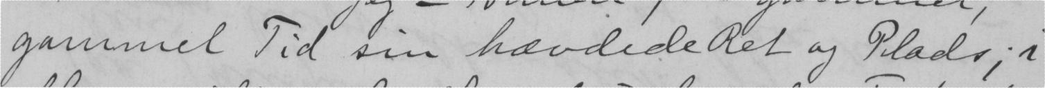gammel Tid sin hævdede Ret og Plads; i
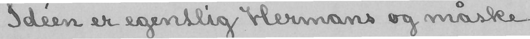Idéen er egentlig Hermans og måske
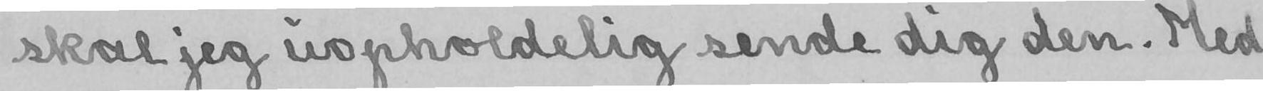skal jeg uopholdelig sende dig den. Med
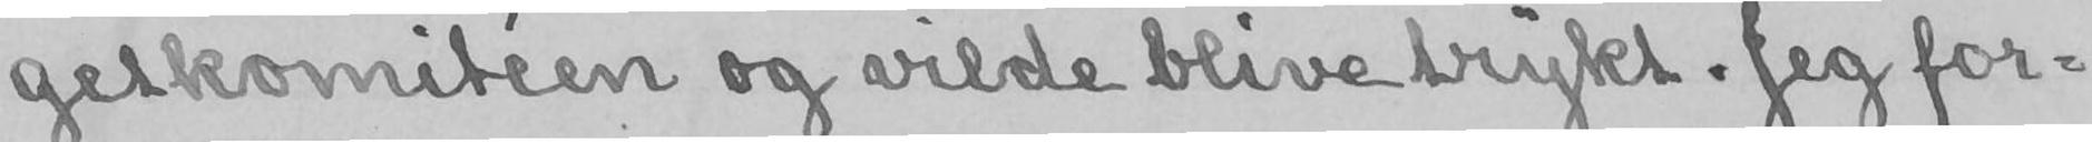getkomitéen og vilde blive trykt. Jeg for-
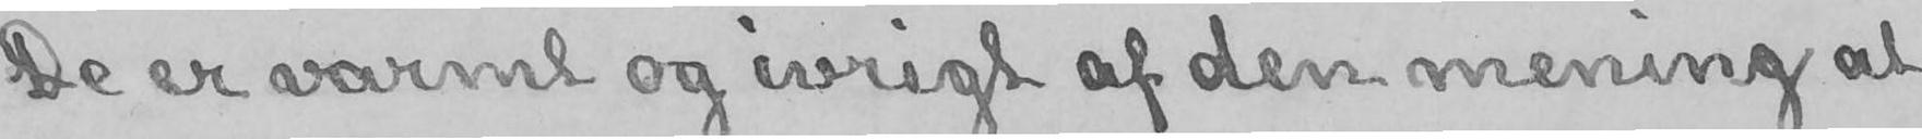De er varmt og ivrigt af den mening at
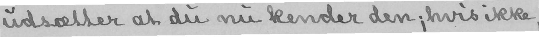udsætter at du nu kender den; hvis ikke,
